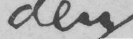den
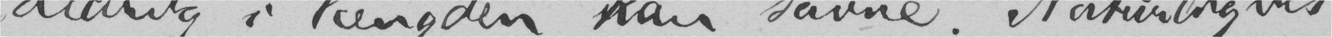aldrig i længden kan savne. Naturligvis
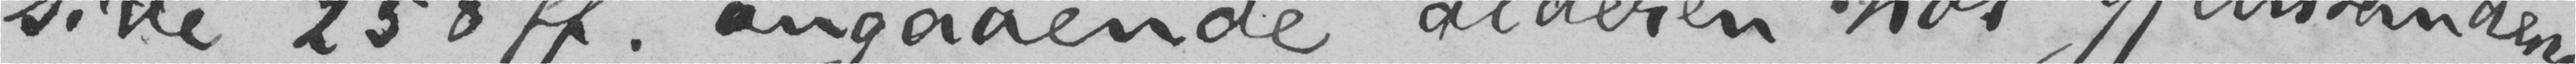side 258 ff. angaaende alderen hos gjenstandene
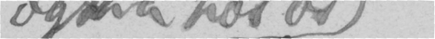ogsaa hos os
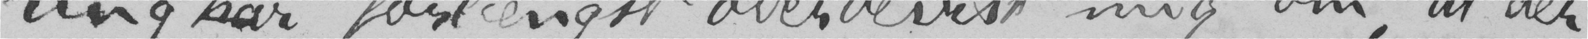ring har forlængst overbevist mig om, at der
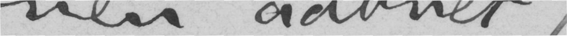nen aabnet
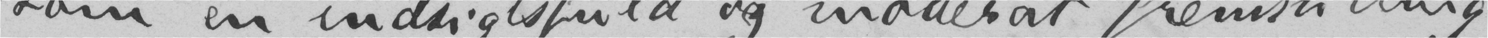som en indsigtsfuld og moderat fremstilling
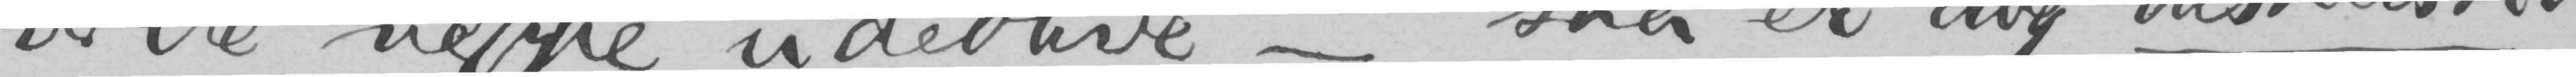ville neppe udeblive - , saa er dog diskussio-
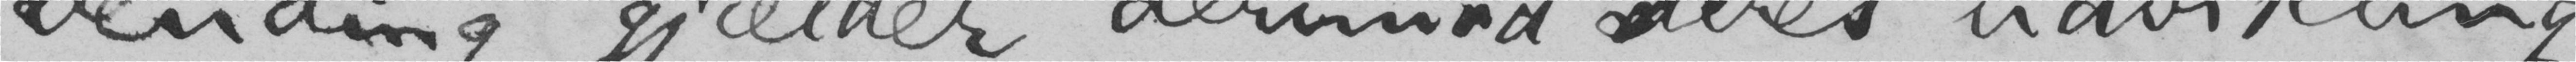vending gjælder derimot Deres udvikling
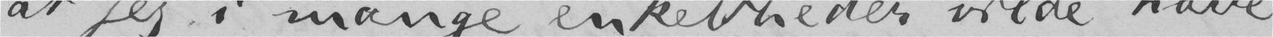at jeg i mange enkeltheder vilde have
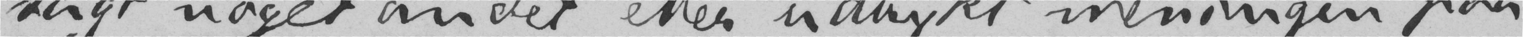sagt noget andet eller udtrykt meningen paa
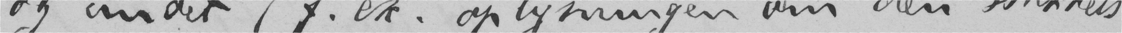og andet (f.ex. oplysningen om den stakkels
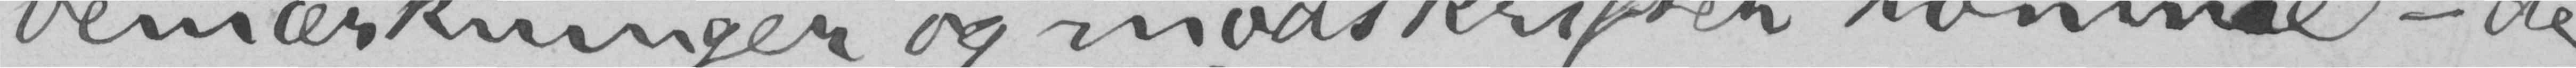bemærkninger og modskrifter komme - de
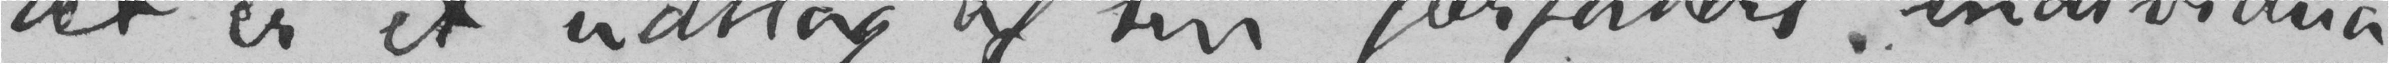det er et udslag af sin forfatters individua-
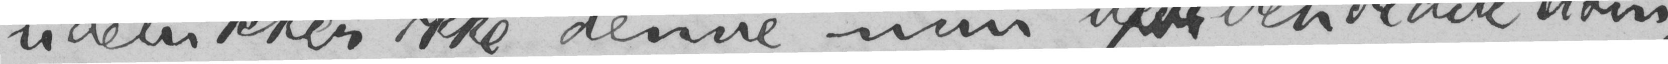udelukker ikke denne min uforbeholdne dom,
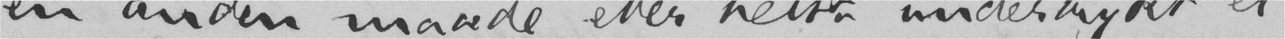en anden maade eller helst undertrykt et
